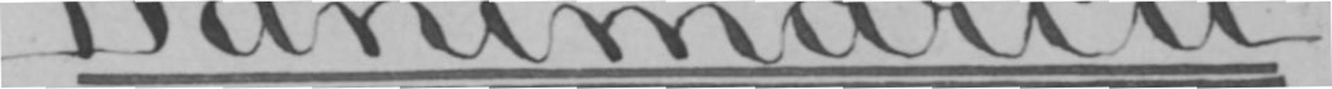(Danimarca.)
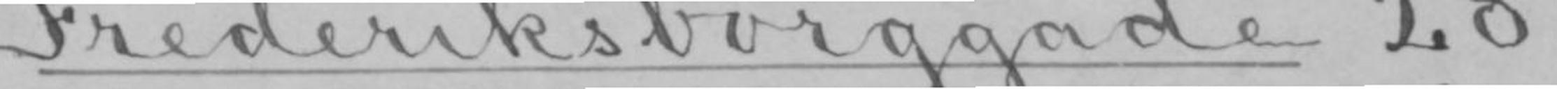Frederiksborggade 28.
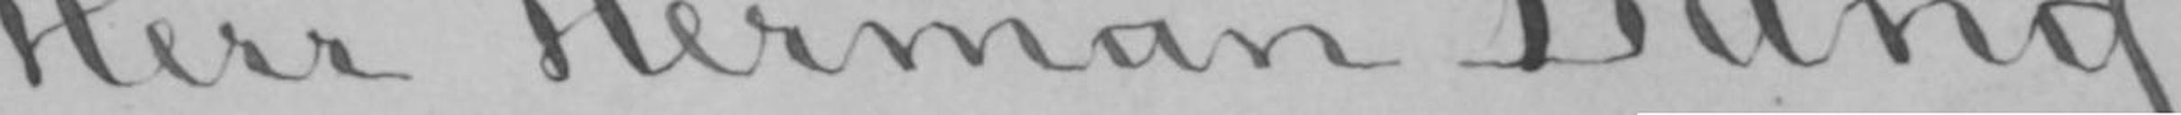Herr Herman Bang.
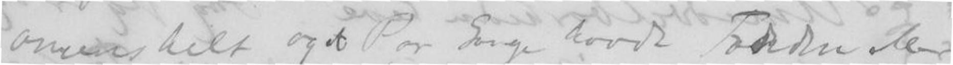amenhelt og et Par Gange havdt Fødder eller
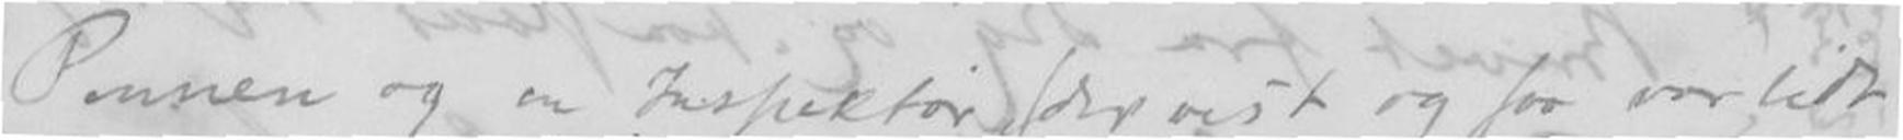Pennen og en Indspektør (der vist ogsaa var lidt
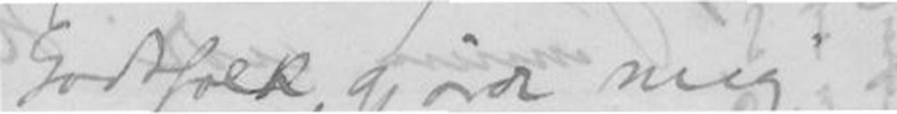Godtfolk, gjorde mig
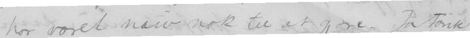har været naiv nok til at gjøre for tænk
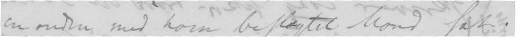en anden med horn beskytet Mand sat.
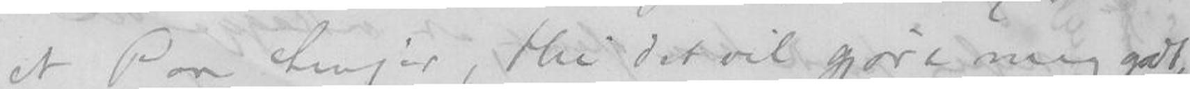et Par Linjer, thi det vil gjøre mig godt
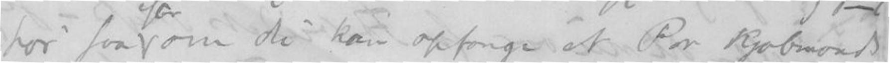hør saa om du kan opfange et Par Kjøbmands
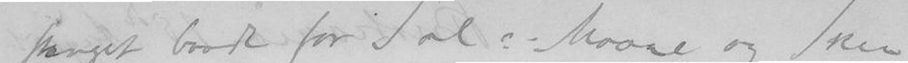og stanget baade for Sol - Maane og Skin
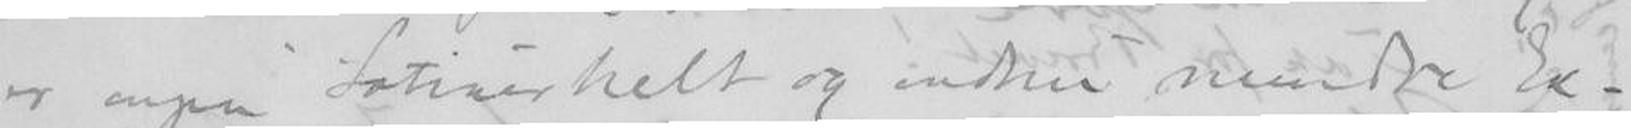er ingen Latinerhelt og endnu mindre Ex-
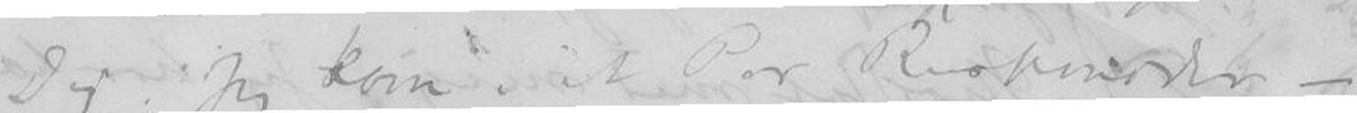Dig. Jeg kom. Et Par Russemøder -
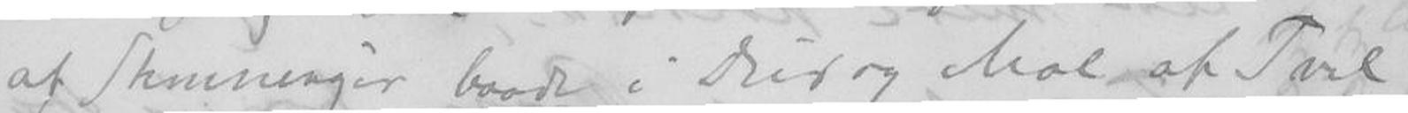af Stemninger baade i Dur og Mol, af Tvil
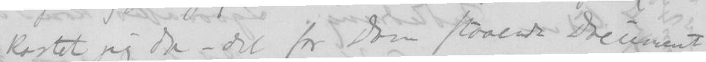kastet jeg da - det for Din staaende Dreument
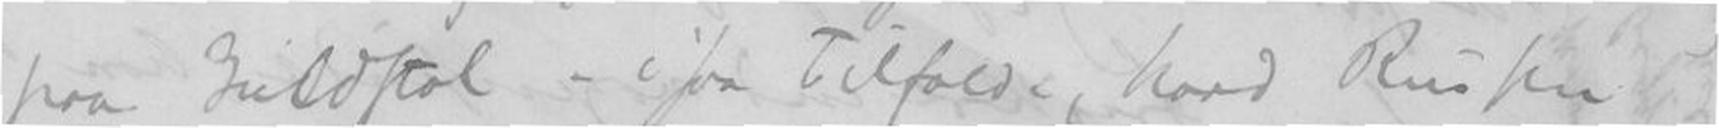mig paa Guldstol - isaa Tilfælde, hvad Russen
